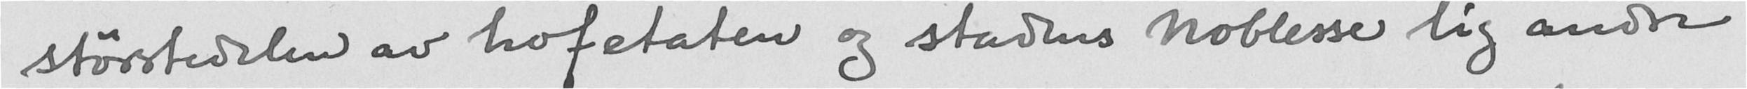størstedelen av hofetaten og stadens noblesse lig andre
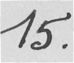15.
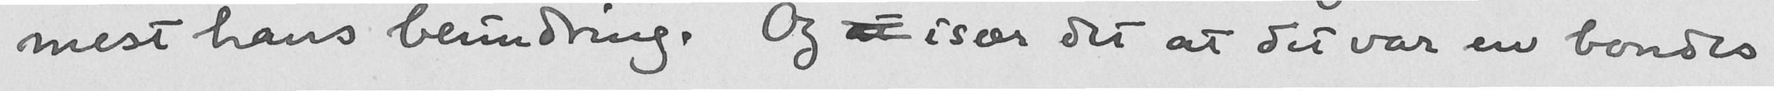mest hans beundring. Og at især det at det var en bondes
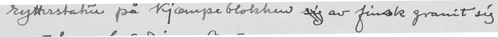rytterstatue på kjæmpeblokken sig av finsk granit sig
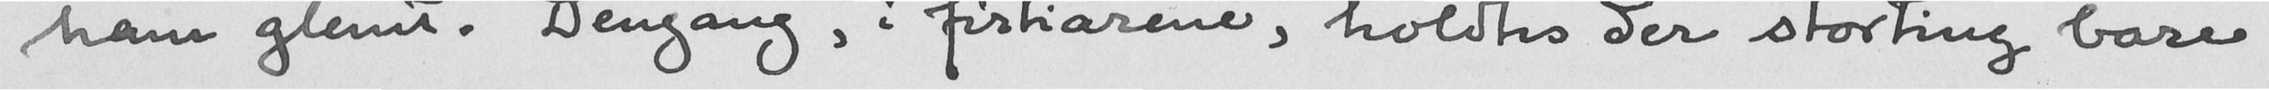ham glemt. Dengang, i firtiårene, holdtes der storting bare
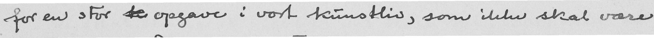for en stor re opgave i vort kunstliv, som ikke skal være
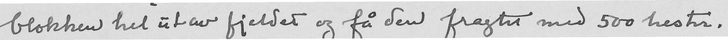blokken hel ut av fjeldet og få den fragtet med 500 hester.
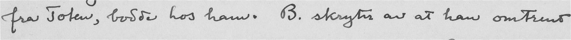fra Toten, bodde hos ham. B. skryter av at han omtrent
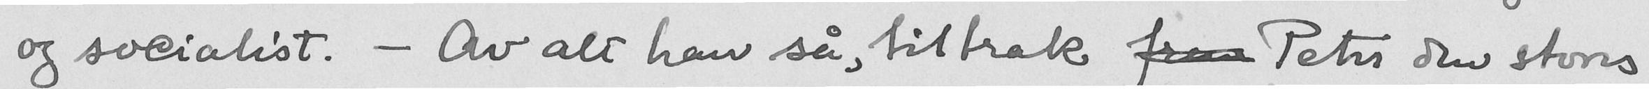og socialist. - Av alt han så, tiltrak frem Peter den stores
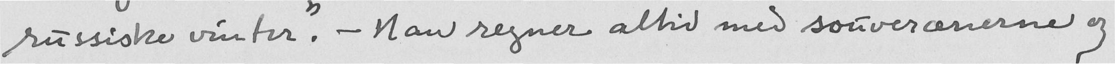russiske vinter". - Han regner altid med souverænerne og
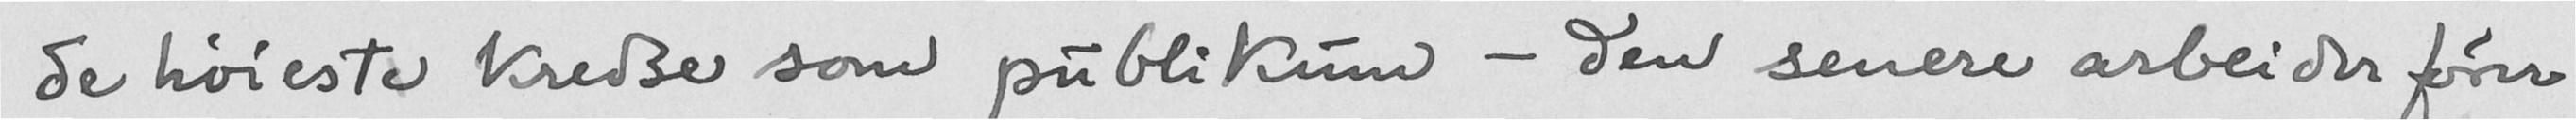de høieste kredse som publikum - den senere arbeider fører
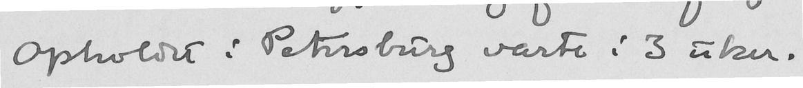Opholdet i Petersburg varte i 3 uker.
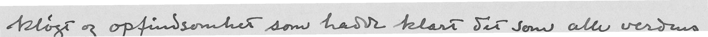kløgt og opfindsomhet som hadde klart det som alle verdens
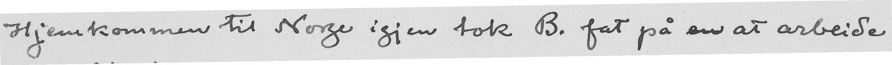Hjemkommen til Norge igjen tok B. fat på en at arbeide
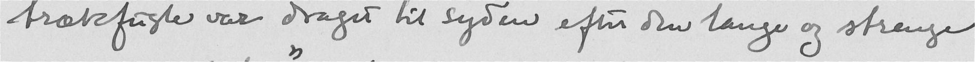trækfugle var draget til syden efter den lange og strenge
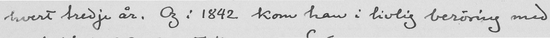hvert tredje år. Og i 1842 kom han i livlig berøring med
